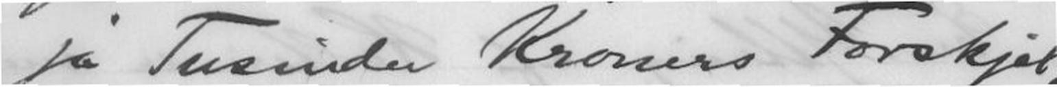ja Tusinder Kroners Forskjel ikke
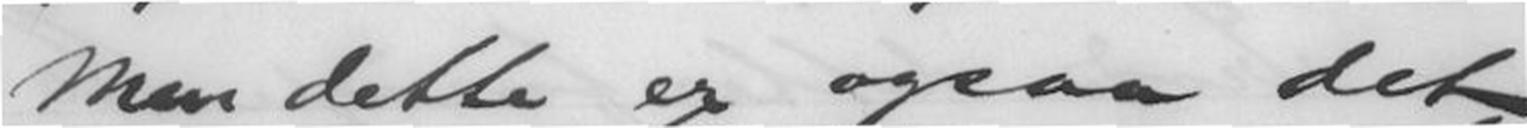Men dette er ogsaa det
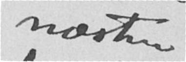næsten
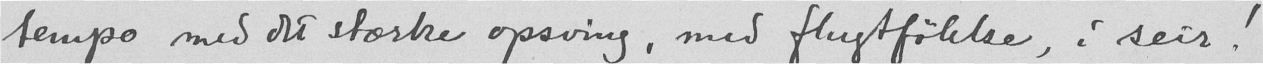tempo med det stærke opsving, med flugtfølelse, i seir!
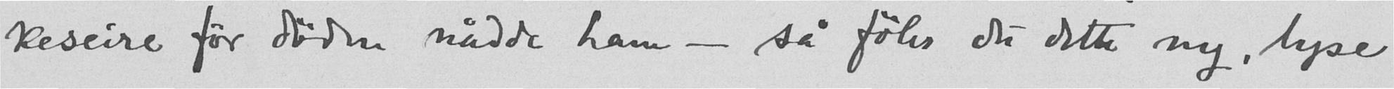keseire før døden nådde ham - så føler du dette ny, lyse
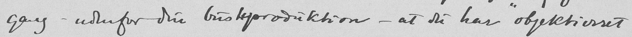gang - udenfor din bustsproduktion - at du har "objektiveret"
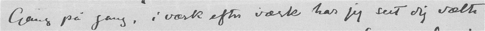Gang på gang, i værk efter værk har jeg seet dig vælte
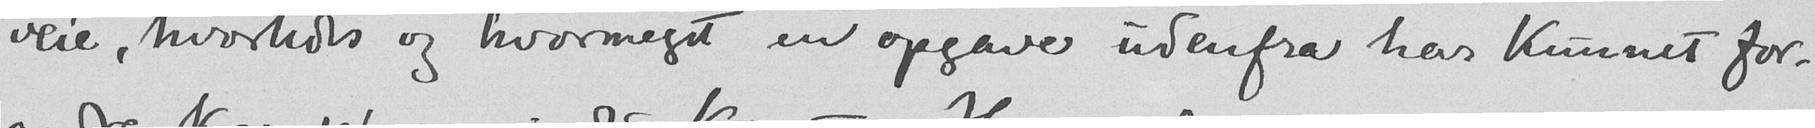veie, hvorledes og hvormeget en opgave udenfra har kunnet for-
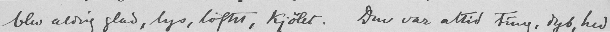blev aldrig glad, lys, løftet, kjølet. Den var altid tung, dyb, hed
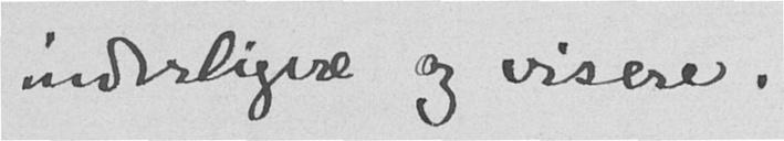inderligere og visere.
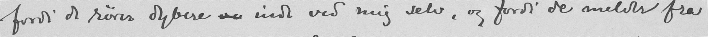fordi de rører dybere ve inde ved mig selv, og fordi de melder fra
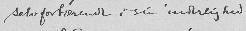selvfortærende i sin inderlighed
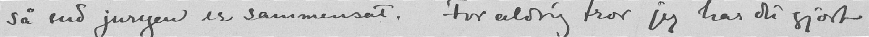så end juryen er sammensat. For aldrig tror jeg har de gjort
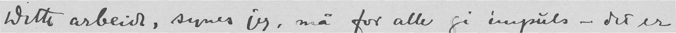Dette arbeide, synes jeg, må for alle gi impuls - det er
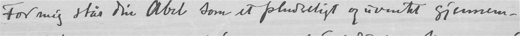For mig står din Abel som et pladseligt og uventet gjennem-
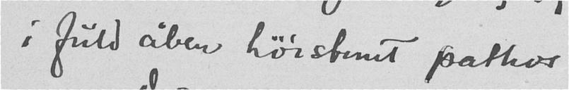i fuld åben høistemt pathos
